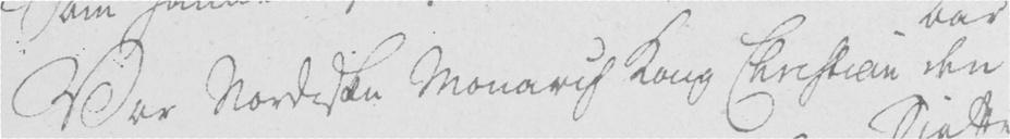Vor Nordiske Monarch Kong Christian den
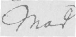Mod
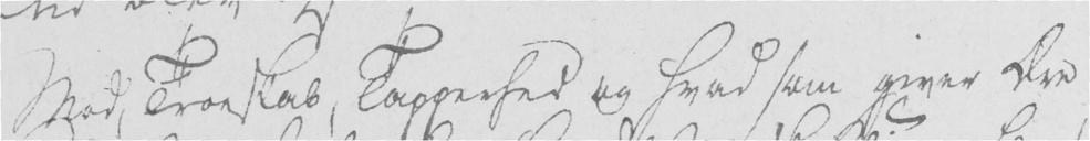Mod, Troeskab, Tapperhed og hvad som giver Ære
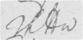dette
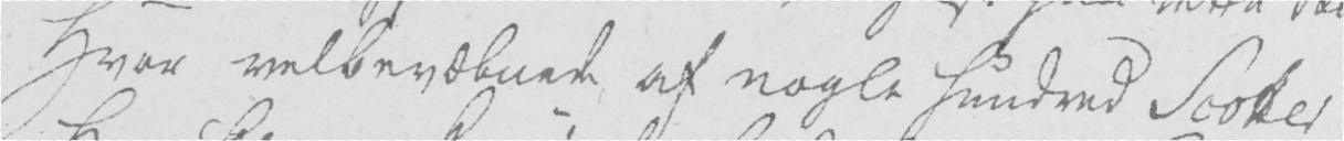Hvor velbevæbnede af nogle hundred Scotter
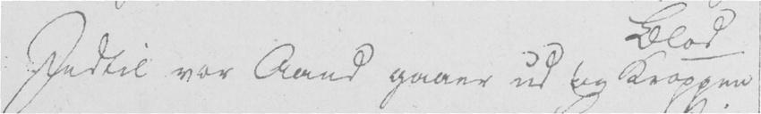Indtil vor Aand gaaer ud og Kroppen
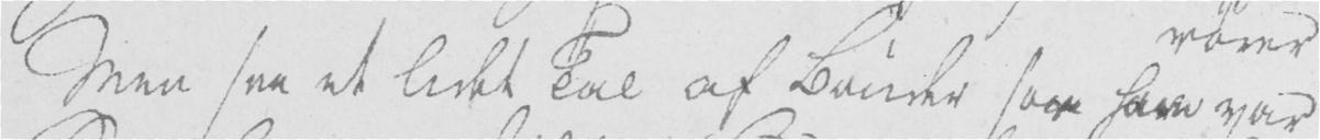Men see et lidet Tal af Bønder for ham var
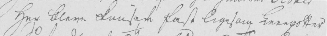Her bleve knusede fast ligesom Leerpotter
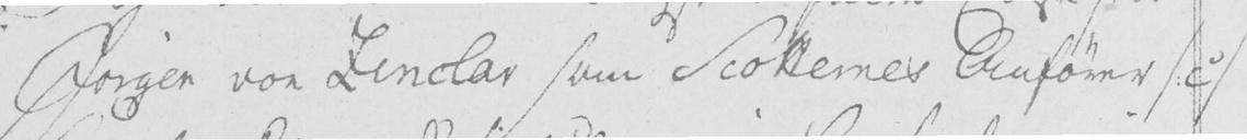Jørgen von Zinclar som Scotternes Anfører /: c /
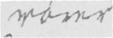rører
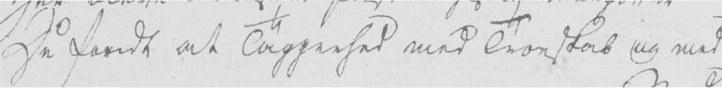De fandt at Tapperhed med Troeskab og med
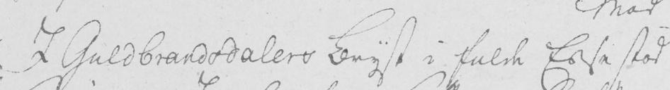I Guldbrandsdalers Bryst i fulde Esse stod
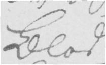Blod
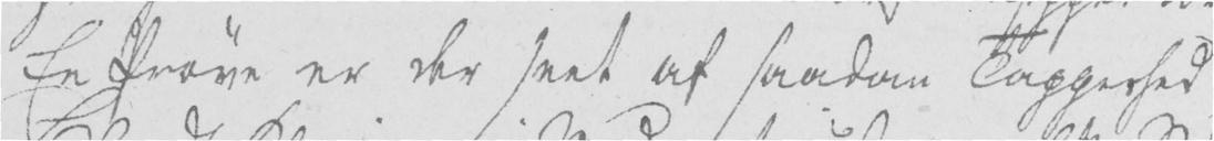En Prøve er der seet af saadan Tapperhed
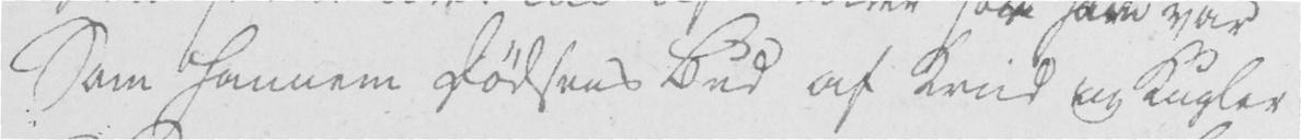Som hannem Dødsens bud af Krud og Kugler
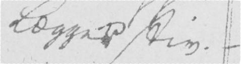lægges stiv. -
